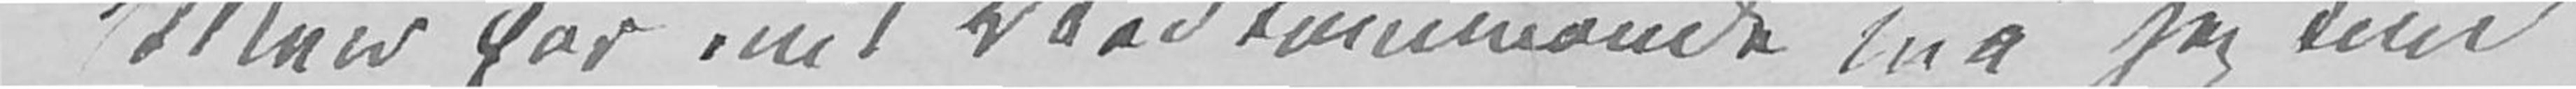Men for mit Vedkommende må jeg kun
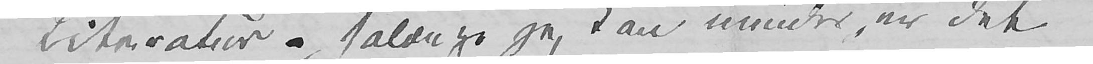Literatur – sålænge jeg kan mindes, er det
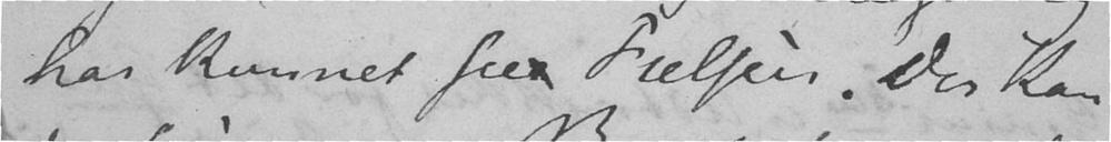har kunnet see Frelsen. Der kan
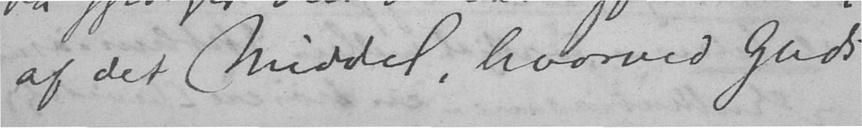af det Middel, hvorved Guds
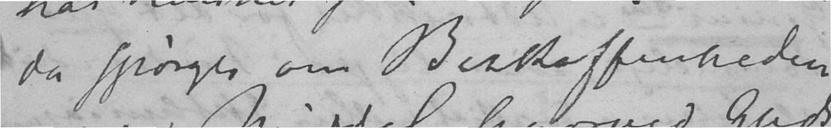da spørges om Beskaffenheden
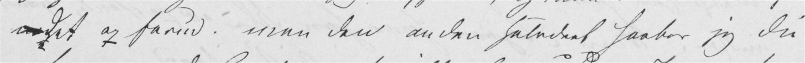nodet op forud. men den anden Halvdeel haaber jeg Du
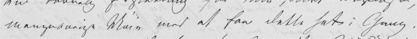mangeaarige Møie med at faa dette sat i Gang.
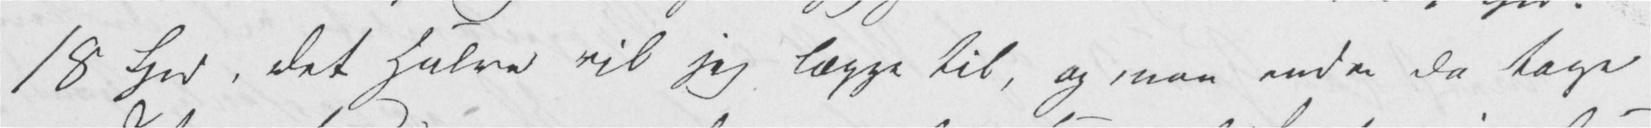18 Spd, det Halve vil jeg lægge til, og maa enda da tage
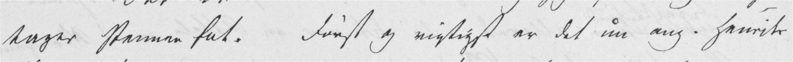tager Pennen fat. Først og vigtigst er det nu ang. Henriks
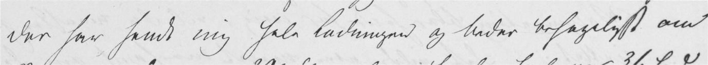der har sendt mig hele Ladningen og beder behageligst om
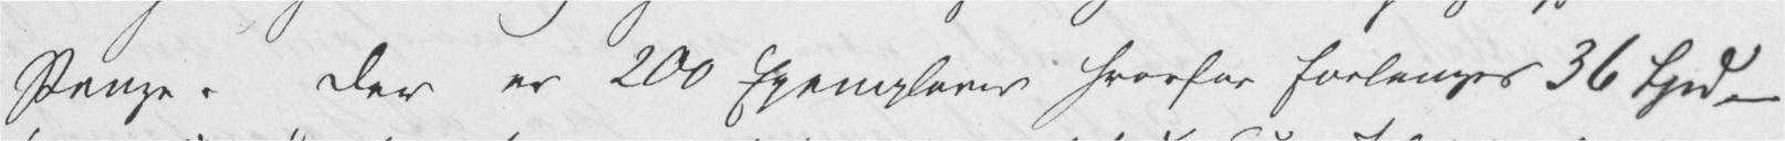Penge. Der er 200 Exemplarer hvorfor forlanges 36 Spd, –
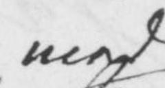med
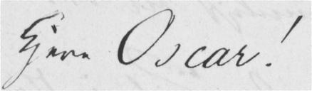Kjere Oscar!
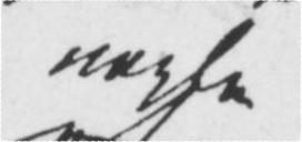nogle
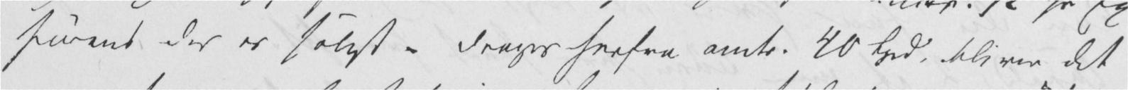førend der er solgt – drages herfra omtr. 40 Spd, bliver det
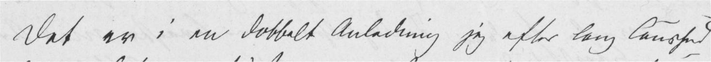Det er i en dobbelt Anledning jeg efter lang Taushed
